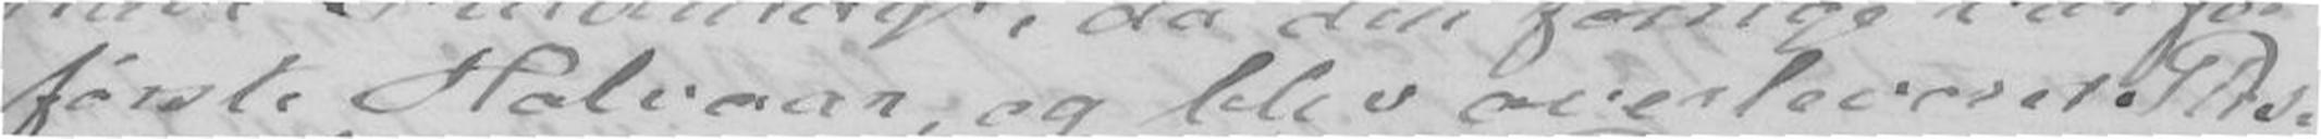første Halvaar; og blev overleveret Ples-
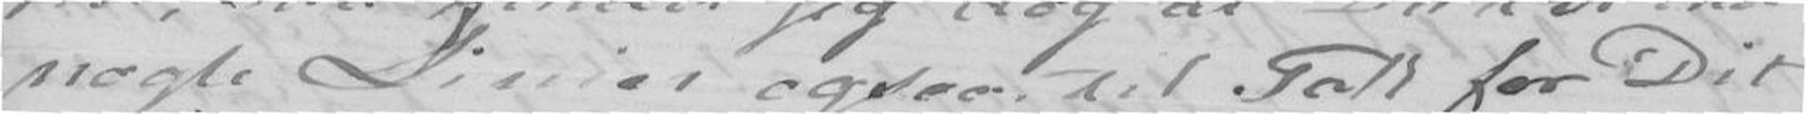nogle Linier ogsaa til Tak for Dit
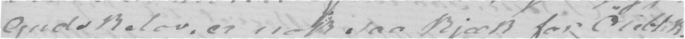Gudskelov er nok saa kjæk for Øieblik-
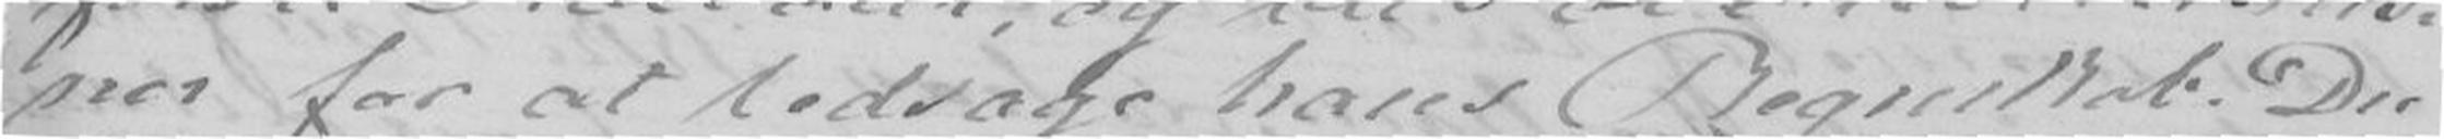ner for at ledsage hans Regnskab. Du
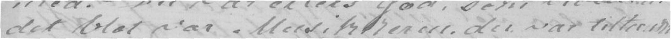det blot var Musikkerne der var tiltænkt
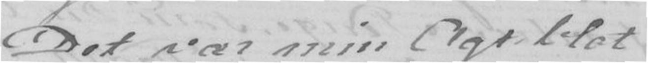Det var min Agt blot
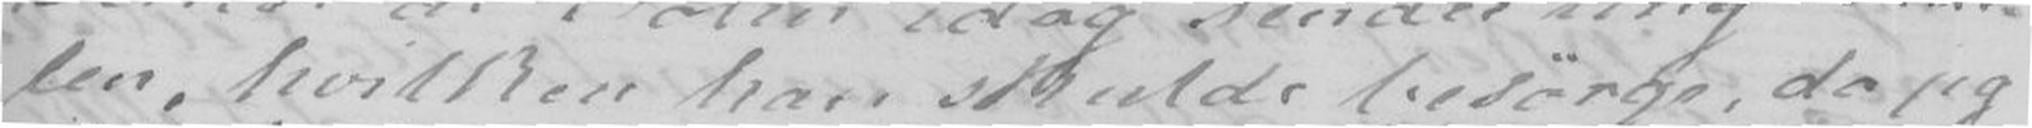len, hvilken han skulde besørge, da jeg
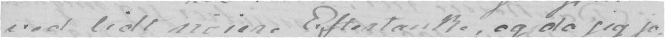ved lidt nøiere Eftertanke, og da jeg jo
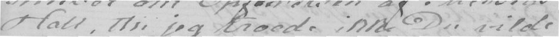Hall, thi jeg troede ikke Du vilde
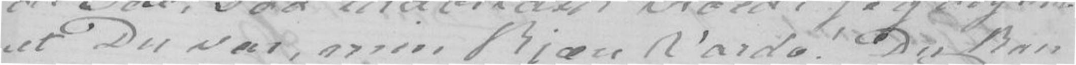at Du var, min kjære Vardo! Du kan
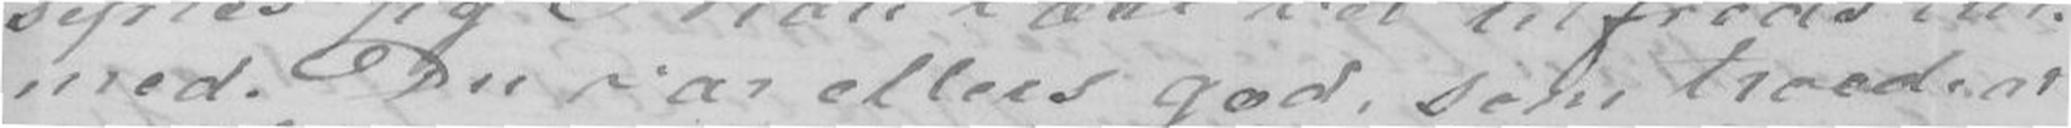med. Du var ellers god, som troede at
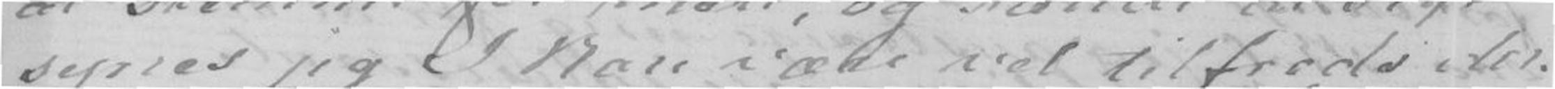synes jeg I kan være vel tilfreds der
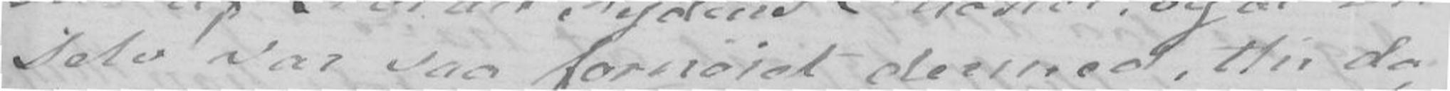selv var saa fornøiet dermed, thi da
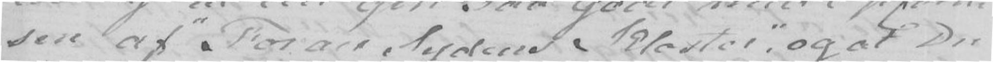sen af "Foran Sydens Kloster", og at Du
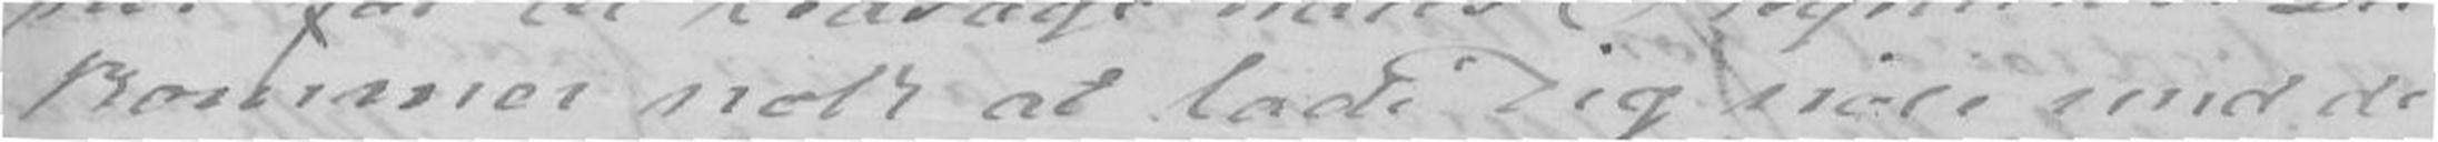kommer nok at lade Dig nøie med de
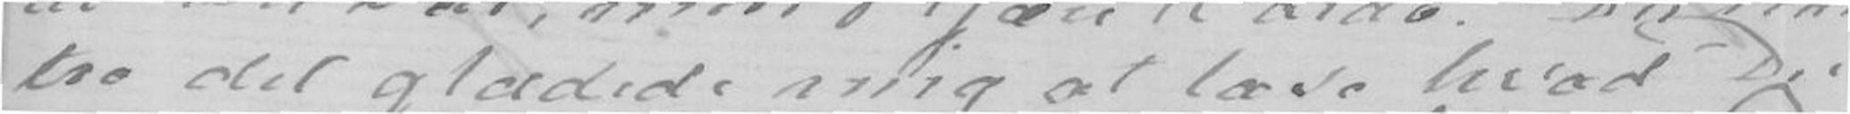tro det glædede mig at læse hvad Du
